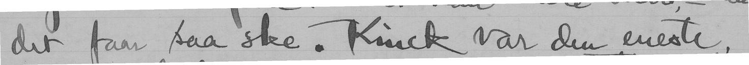det faar saa ske. Kinck var den eneste,
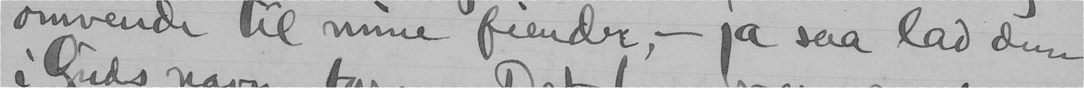omvende til mine fiender - ja saa lad dem
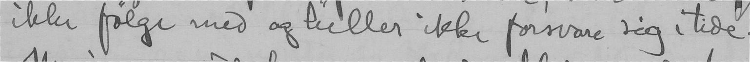ikke følge med og heller ikke forsvare sig i tide.
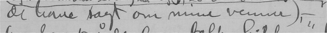de have sagt om mine venner). -
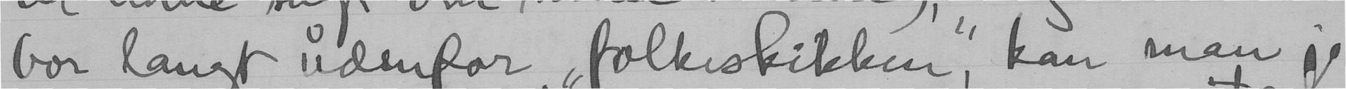bor langt udenfor "folkeskikken", kan man jo
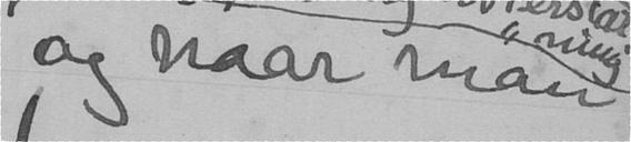og naar man
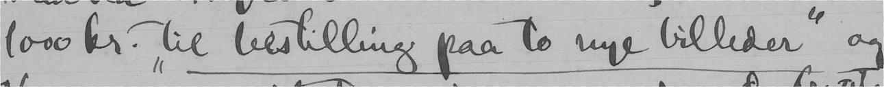1000 kr. "til bestilling paa to nye billeder" og
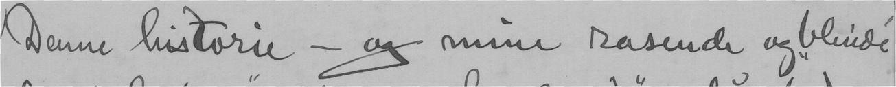Denne historie - og mine rasende og "blinde"
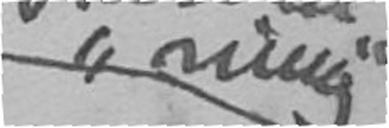ning
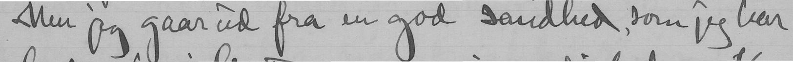Men jeg gaar ud fra en god sandhed, som jeg har
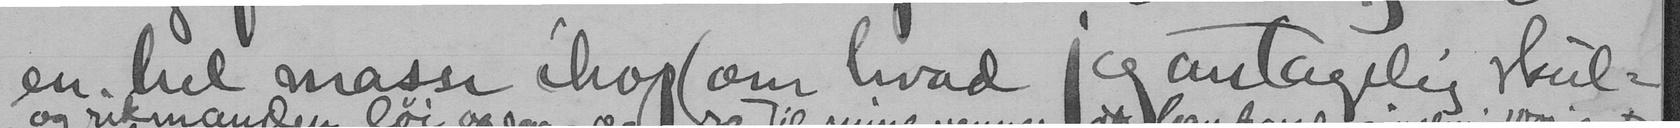en hel masse ihog(om hvad jeg antagelig skul-
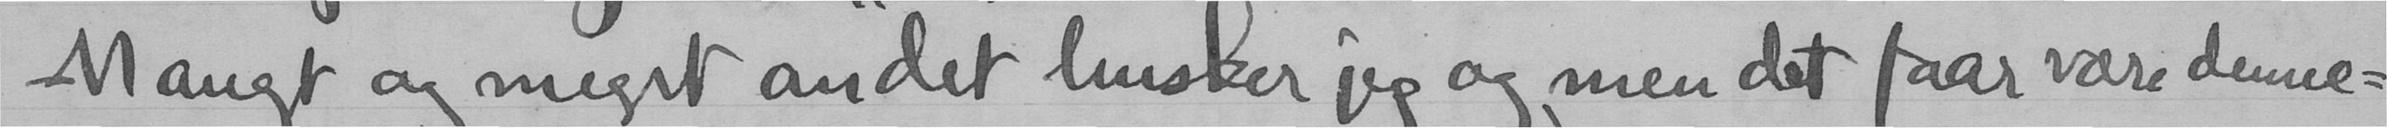Mangt og meget andet husker jeg og, men det faar være denne-
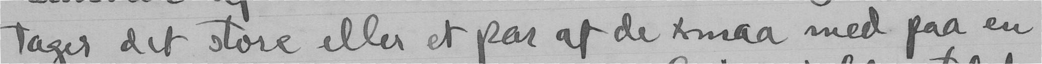tager det store eller et par af de smaa med paa en
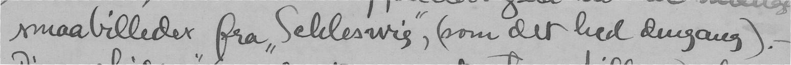smaabilleder fra "Sehleswig", (som det hed dengang).
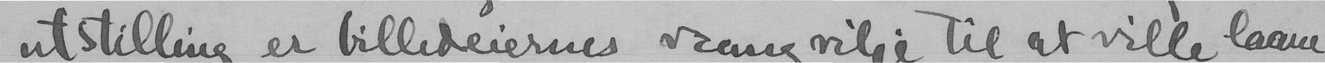utstilling er billedeiernes vrangvilje til at ville laane
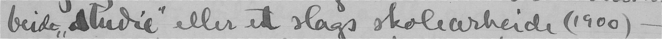beide "studie" eller et slags skolearbeide (1900) -
